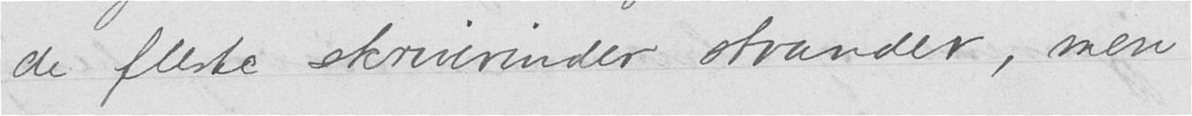de fleste skriverinder strander, men
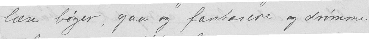læse bøger, gaa og fantasere og drømme
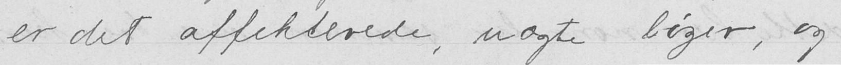er det affekterede, uægte bøger, og
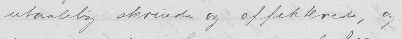utaalelig skruede og affekterede, og
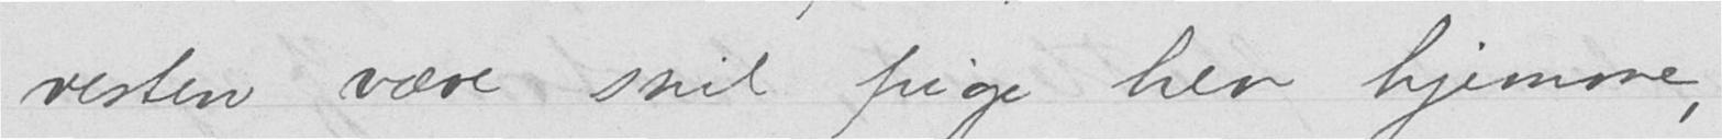resten være snil pige her hjemme,
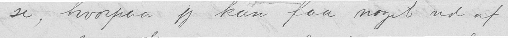se, hvorpaa jeg kan faa noget ud af
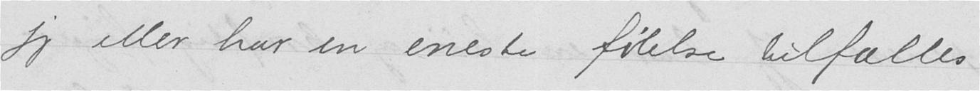jeg eller har en eneste følelse tilfælles
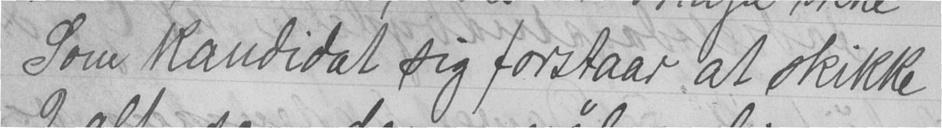Som kandidat sig forstaar at skikke
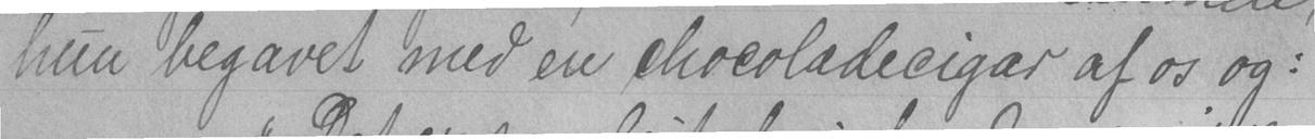hun begavet med en chocoladesigar af os og:
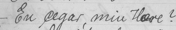- En cegar, min kære?
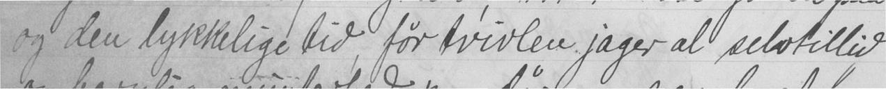og den lykkelige tid, før tvivlen jager al selvtillid
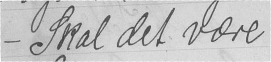- Skal det være
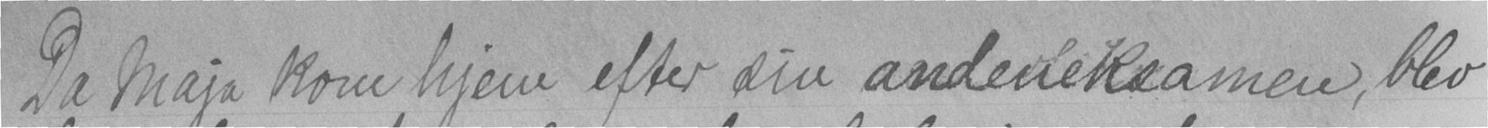Da Maja kom hjem efter sin andeneksamen, blev
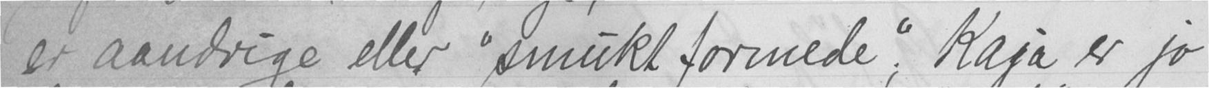er aandrige eller "smukt formede"; Kaja er jo
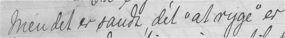Men det er sandt, det "at ryge" er
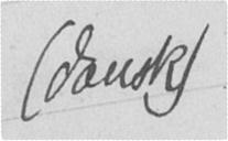(dansk)
